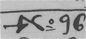No 96
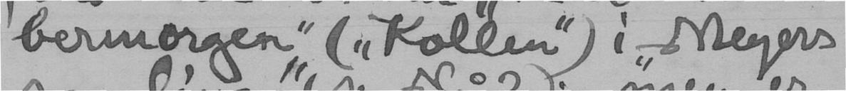bermorgen" ("Kollen") i "Meyers
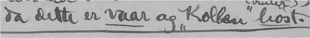da dette er vaar og "Kollen" høst.
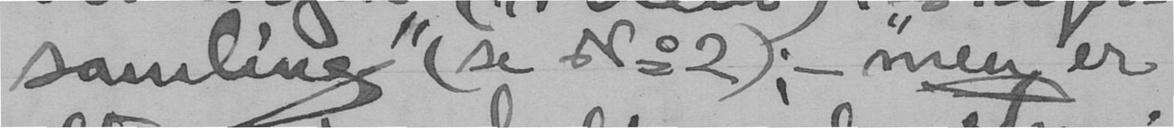samling" (se No 2); - men er
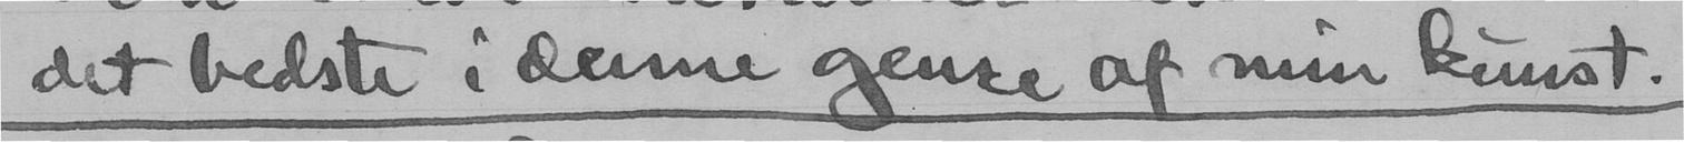det bedste i denne genre af min kunst.
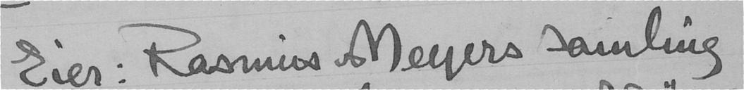Eier: Rasmus Meyers samling
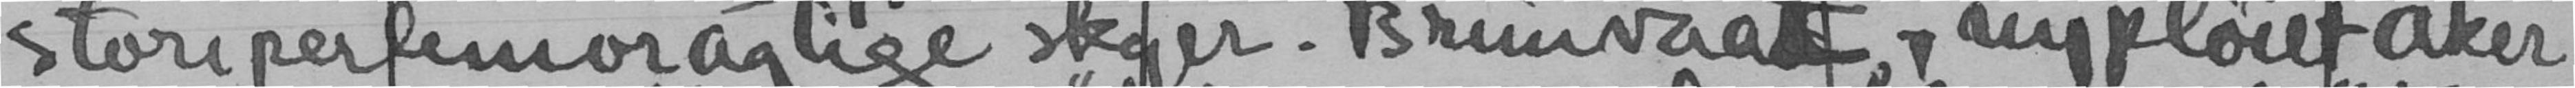storlemoragtige skyer. Brunvaadt nypløiet aker
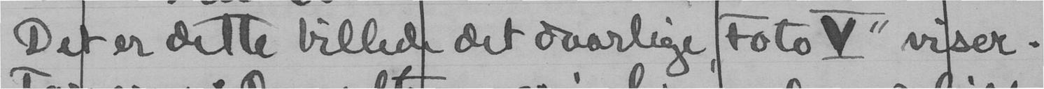Det er dette billedt det daarlige "Foto V" viser.
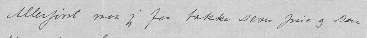Allerførst maa jeg faa takke Deres frue og Dem
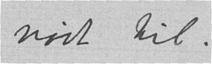nødt til.
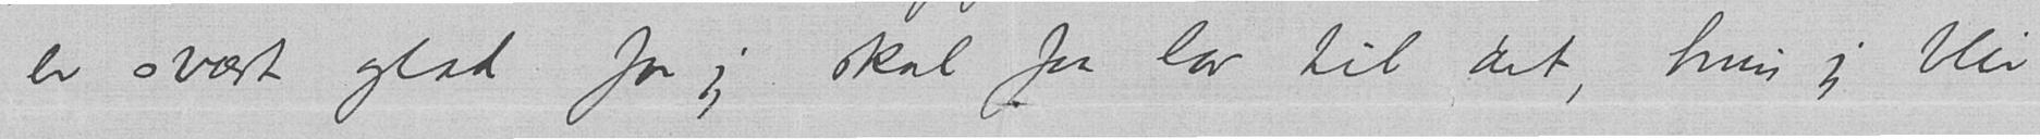er svært glad for jeg skal faa lov til det, hvis jeg blir
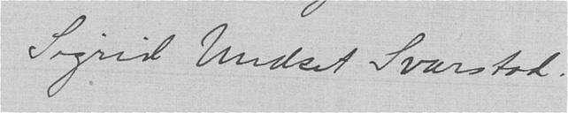Sigrid Undset Svarstad.
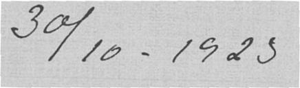30/10-1923
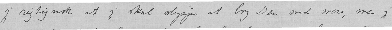jeg rigtignok at jeg skal slippe at bry Dem med mere, men jeg
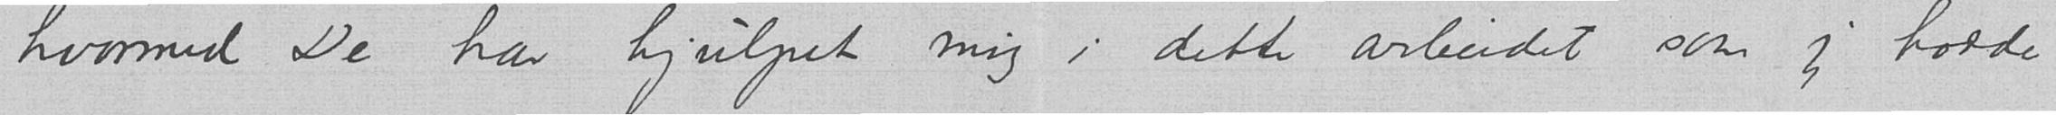hvormed De har hjulpet mig i dette arbeidet som jeg hadde
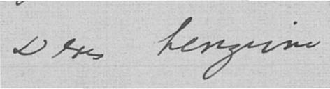Deres hengivne
